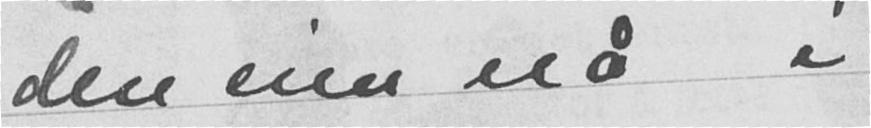den inn nå i
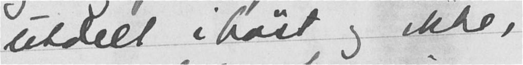utdelt ihøst og ikke,
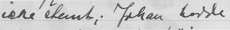ikke stemt; Johan hadde
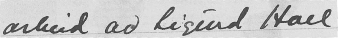arbeid av Sigurd Hoel
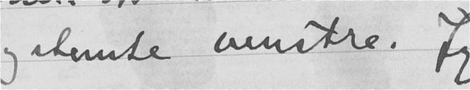og stemte venstre. Jeg
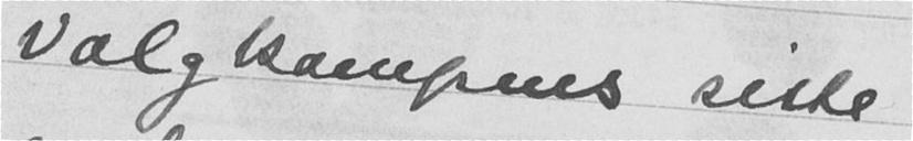valgkampens siste
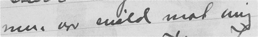men var mild mot mig
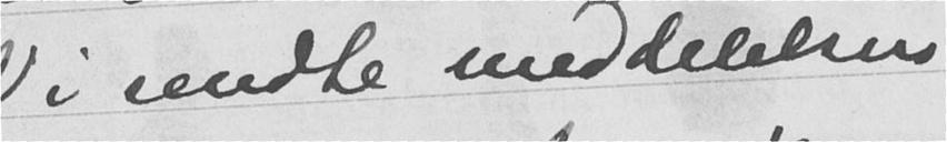Vi sendte meddelelsen
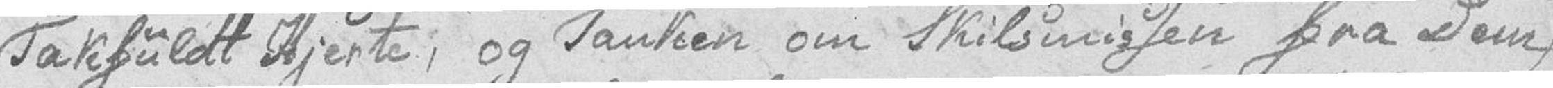Takfuldt Hjerte, og Tanken om Skilsmissen fra Dem,
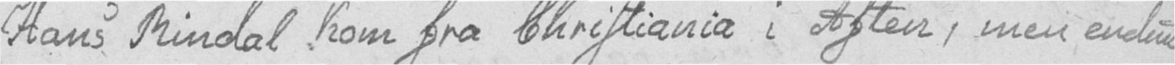Hans Rindal kom fra Christiania i Aften, men endnu
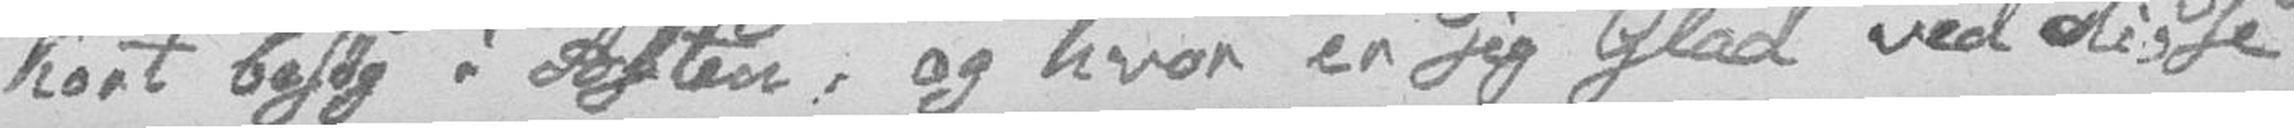kort besøg i Aften, og hvor er jeg Glad ved disse
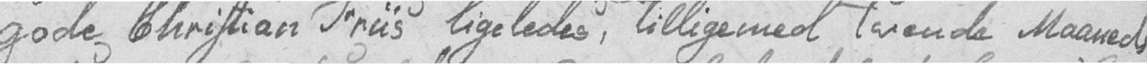gode Christian Friis ligeledes, tilligemed tvende Maaneds
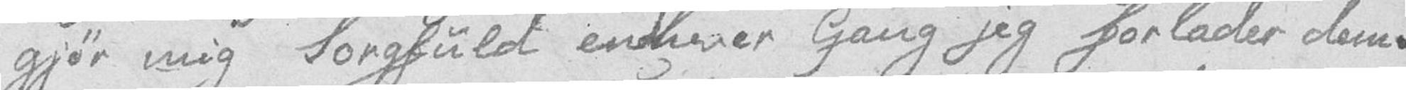gjør mig Sorgfuld endhver Gang jeg forlader dem.
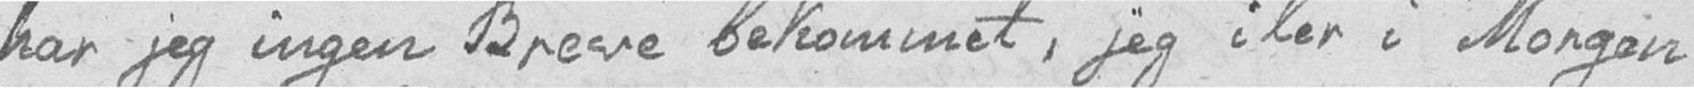har jeg ingen Breve bekommet, jeg iler i Morgen
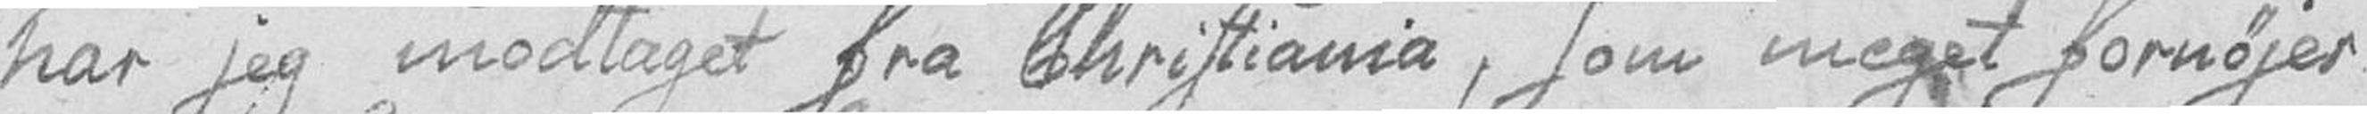har jeg modtaget fra Christiania, som meget fornøjer
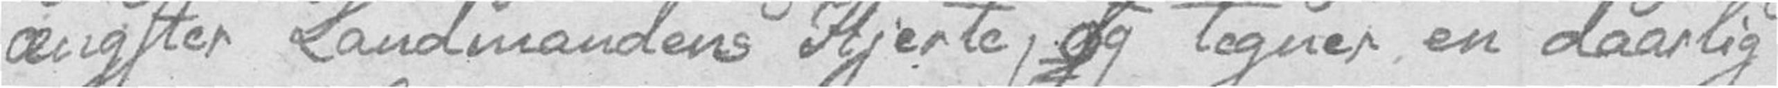ængster Landmandens Hjerte, og tegner en daarlig
